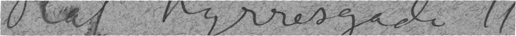Olaf Kyrresgade 11
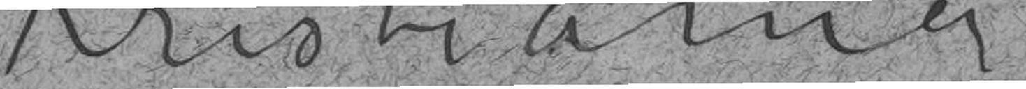Kristiania
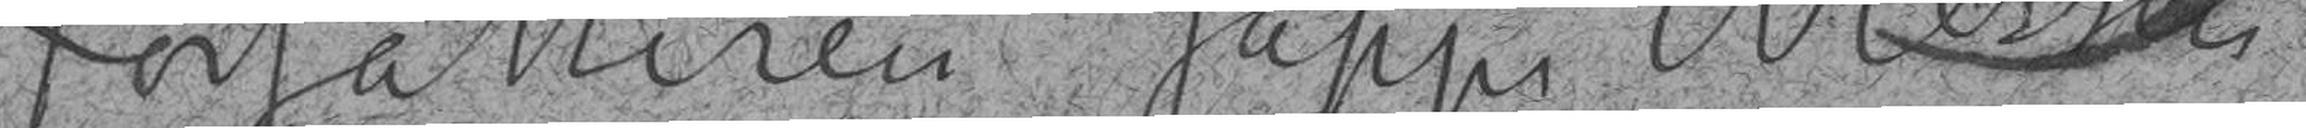Forfatteren Jappe Nilssen
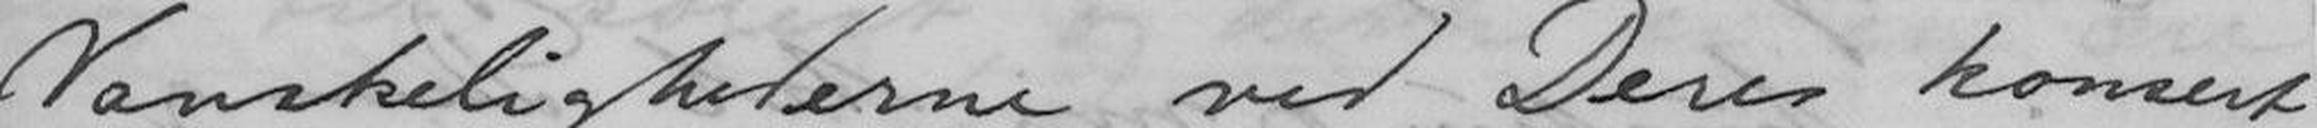Vanskelighederne ved Deres konsert
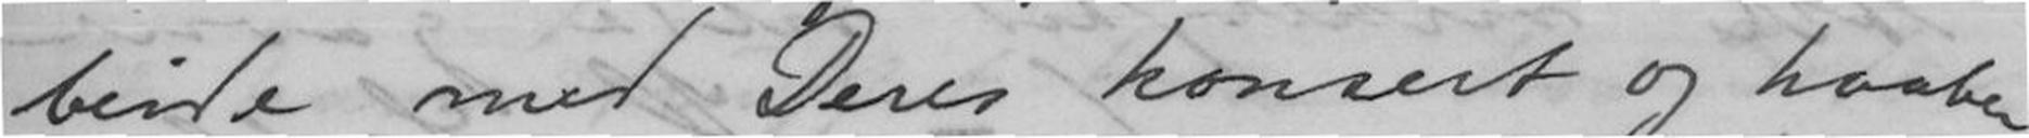beide med Deres konsert og haaber
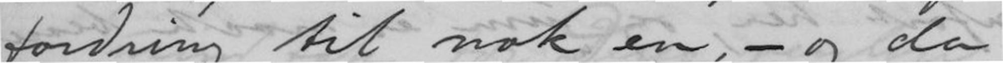fordring til nok en, - og da
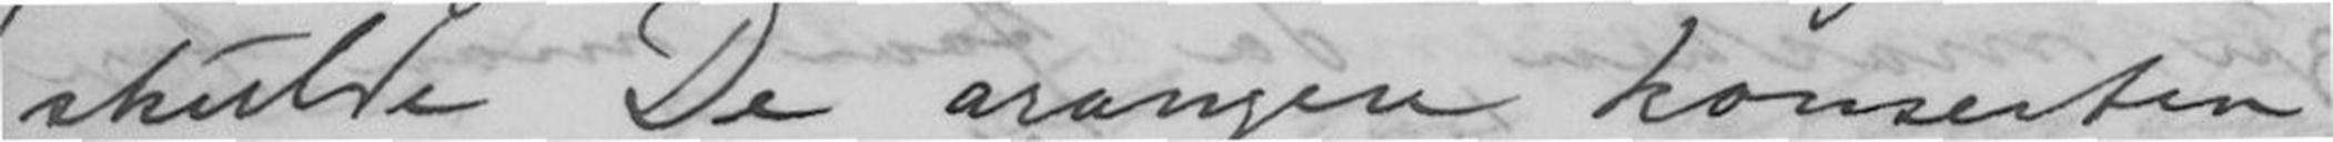skulde De arangere konserten
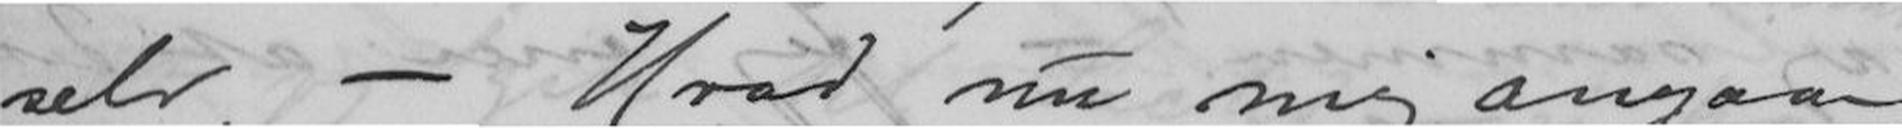selv. - Hvad nu mig angaar,
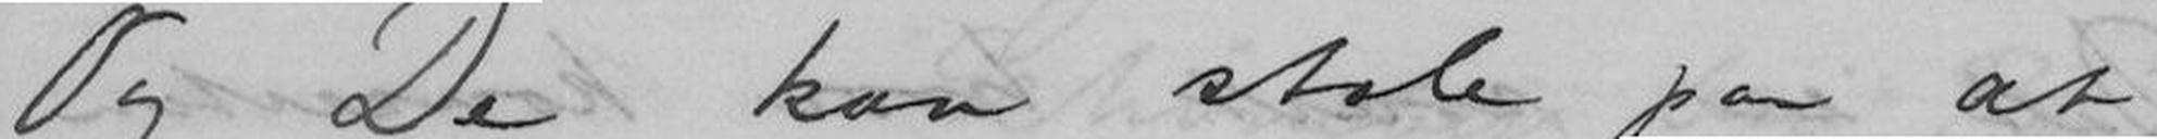Og De kan stole paa at
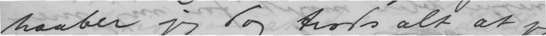haaber jeg dog trods alt at jeg
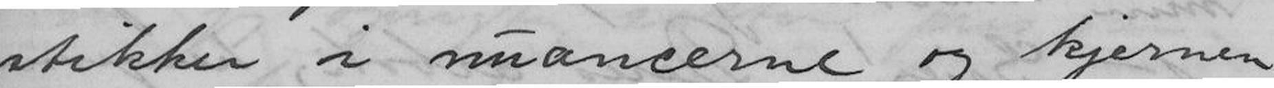stikker i nuancerne og kjernen
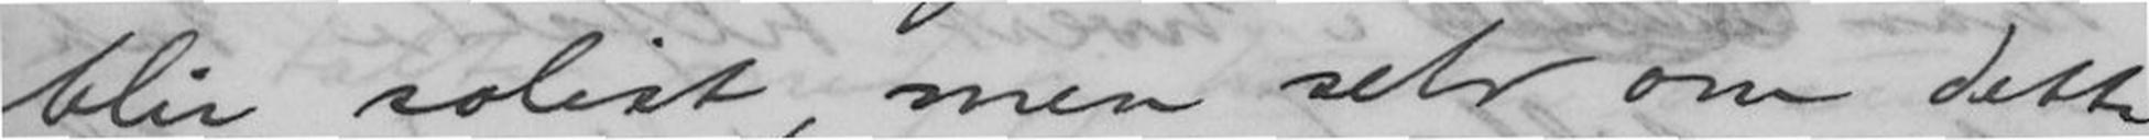blir solist, men selv om dette
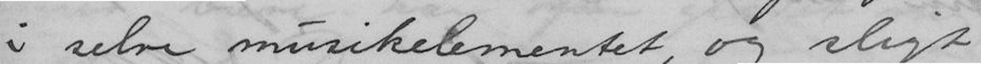i selve musikelementet, og sligt
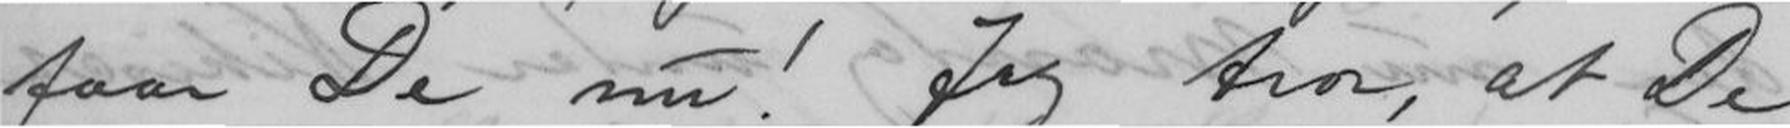faar De nu! Jeg tror, at De
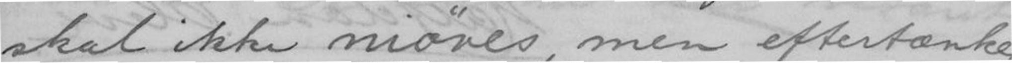skal ikke niøves, men eftertænkes.
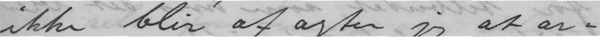ikke blir af agter jeg at ar-
